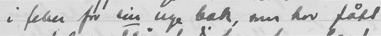i feber for sin nye bok, som har fått
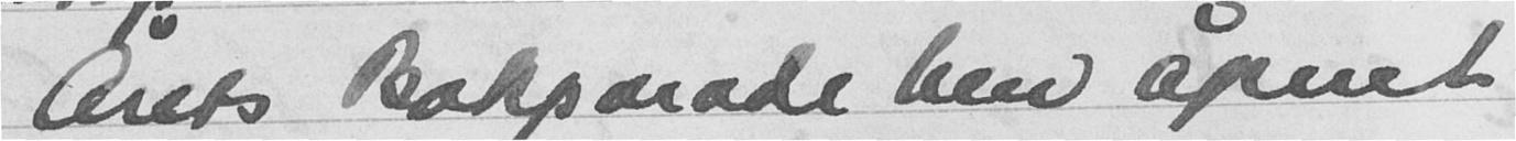Årets Bokparade blev åpnet
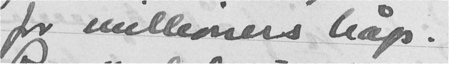for millioners håp.
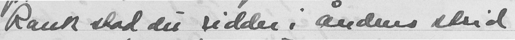Rank stod du ridder i åndens strid
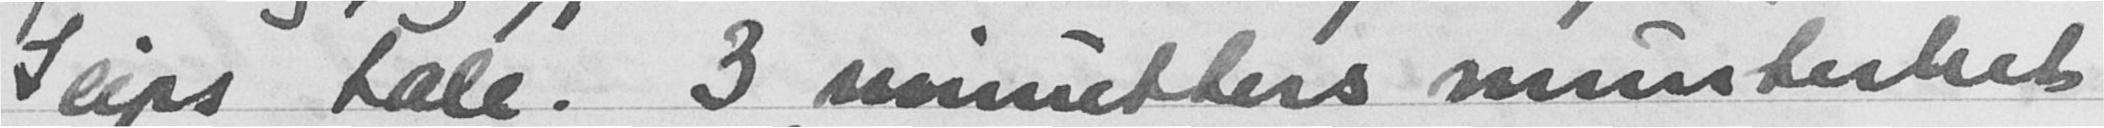Seips tale. 3 minutters munterhet
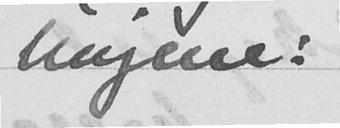linjene:
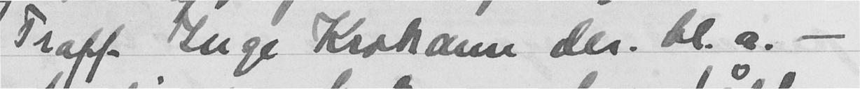Traff Inge Krokann der. bl. a. -
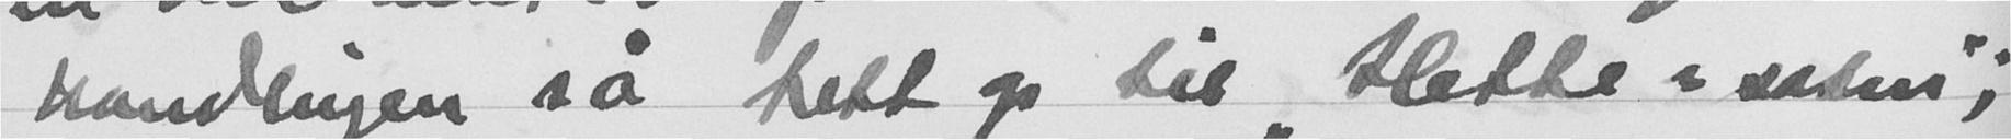handlingen så hett op til "Hetle-saken";
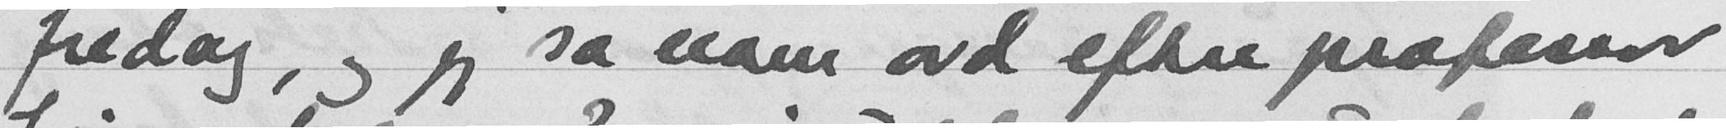fredag, og jeg sa noen ord efter professor
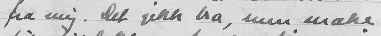fra mig. Det gikk bra, men make
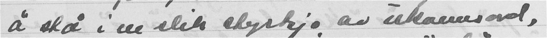å stå i en slik styrtsjø av ukvemsord,
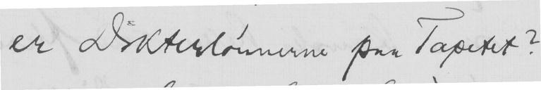er Dikterlønnerne paa Tapetet?
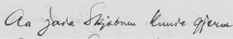Aa jada Skjæbnen kunde gjerne
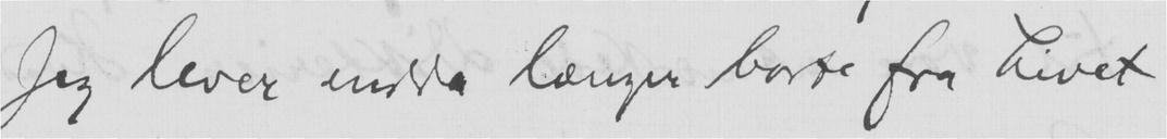Jeg lever endda længer borte fra Livet
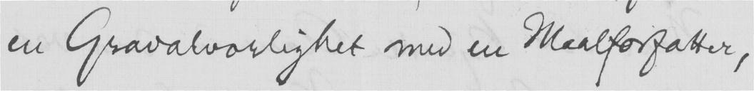en Gravalvorlighet med en Maalforfatter,
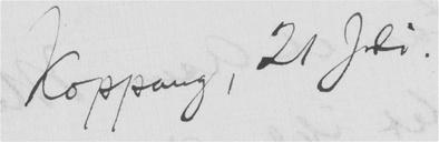Koppang, 21 Juli.
